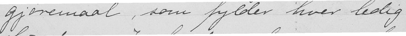gjøremaal, som fylder hver ledig
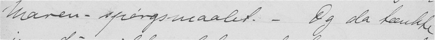Maren-spørgsmaalet.- Og da tænkte
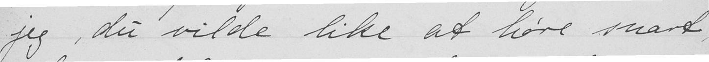jeg, du vilde like at høre snart,
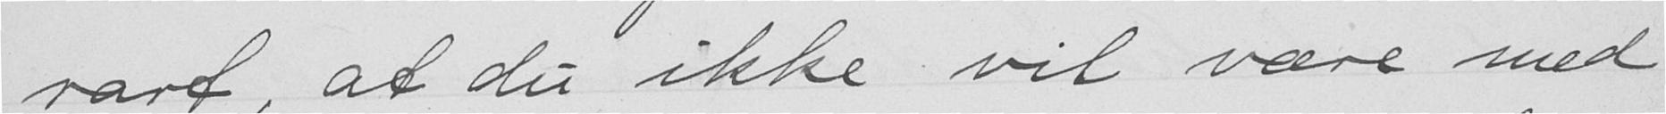rart, at du ikke vil være med
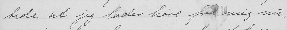tide at jeg lader høre fra mig nu;
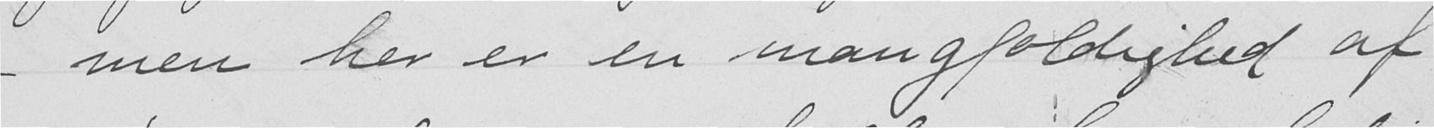- men her er en mangfoldighed af
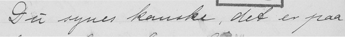Du synes kanske, det er paa
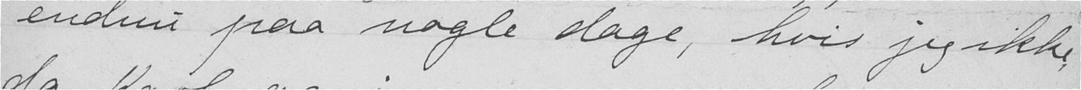endnu paa nogle dage, hvis jeg ikke
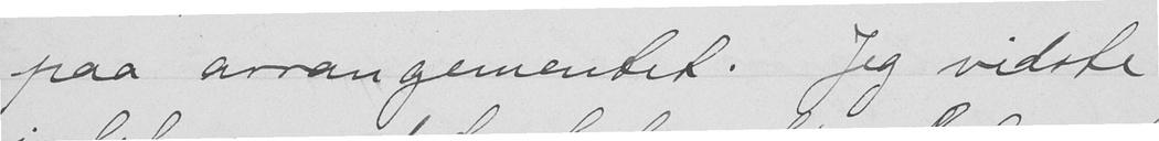paa arrangementet. Jeg vidste
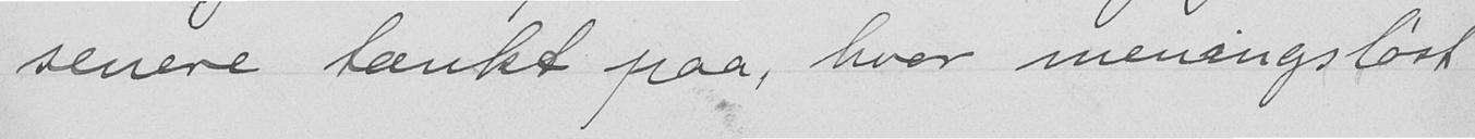senere tænkt paa, hvor meningsløst
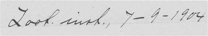Zoot. inst., 7-9-1904
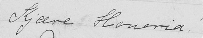Kjære Honoria!
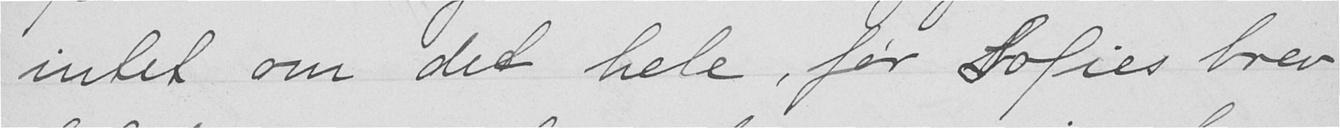intet om det hele, før Sofies brev
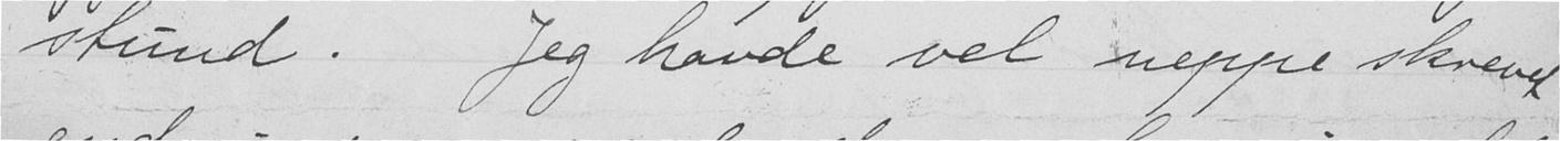stund. Jeg havde vel neppe skrevet
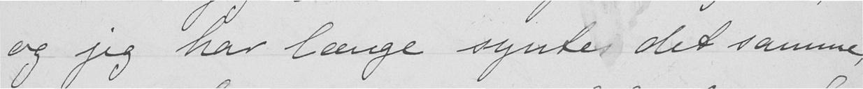og jeg har længe syntes det samme,
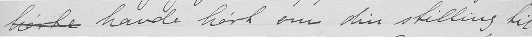hørte havde hørt om din stilling til
